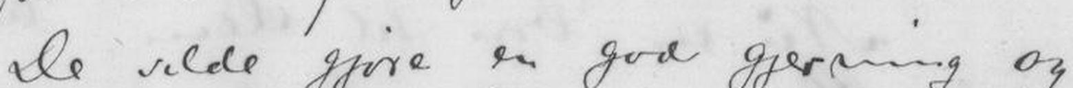De vilde gjøre en god gjerning og
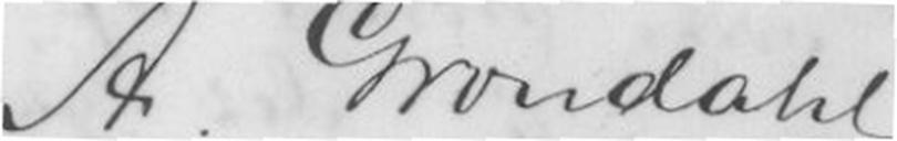A. Grøndahl
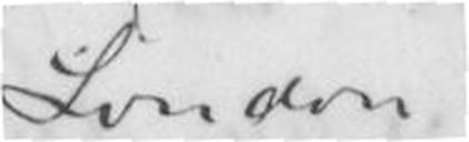London
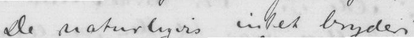De naturligvis intet bryderi
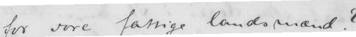for vore fattige landsmænd?
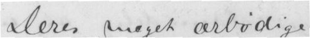Deres meget ærbødige
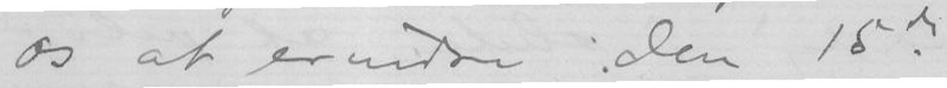os at erindre den 15 de.
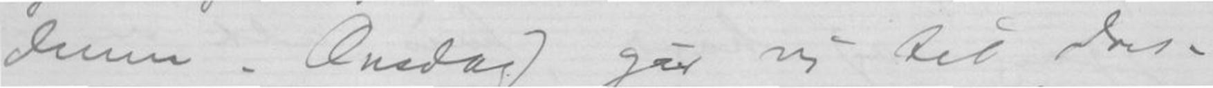denne. Onsdag går vi til Dres-
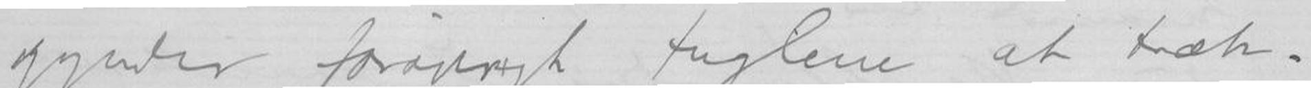gynder forøvrigt fuglene at træk-
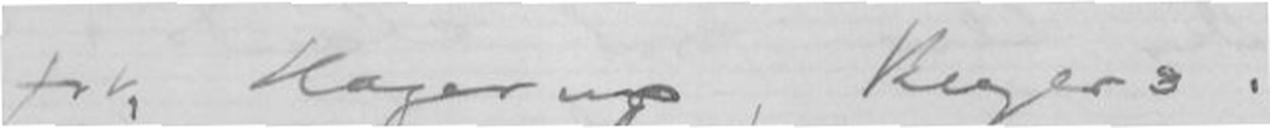frk Hagerup, Beyers.
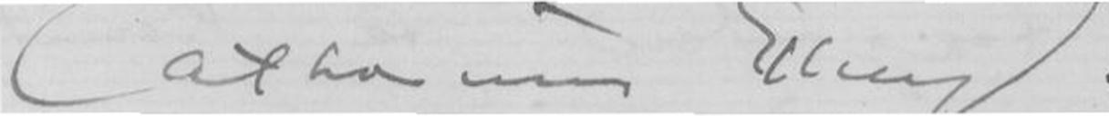Catharinus Elling
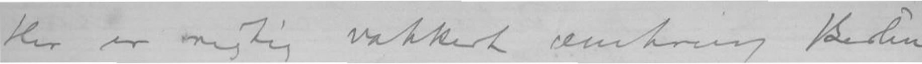Her er rigtig vakkert omkring Berlin.
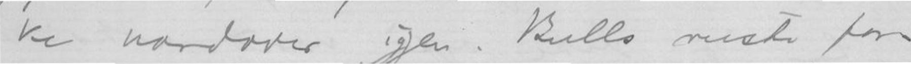ke nordover igjen. Bulls reiste for-
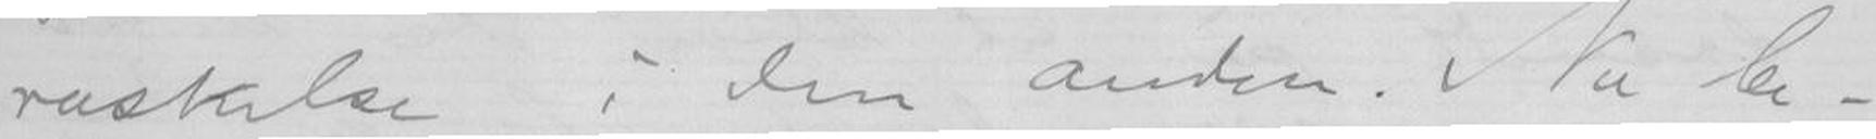raskelse i den anden. Nu be-
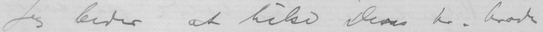Jeg beder at hilse Deres hr. broder
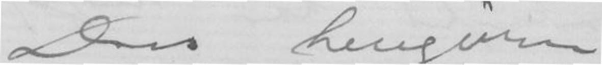Deres hengivne
